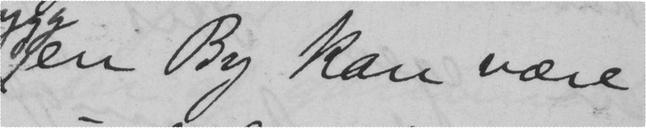en By kan være
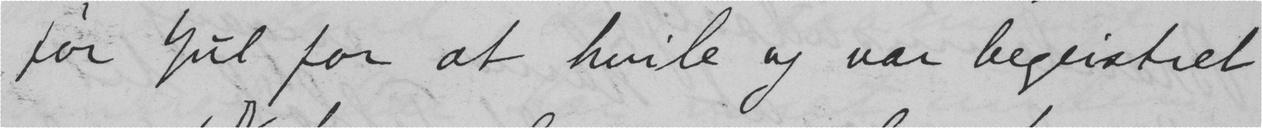før jul for at hvile og var begeistret
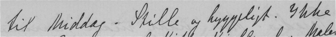til Middag. Stille og hyggeligt. Ikke
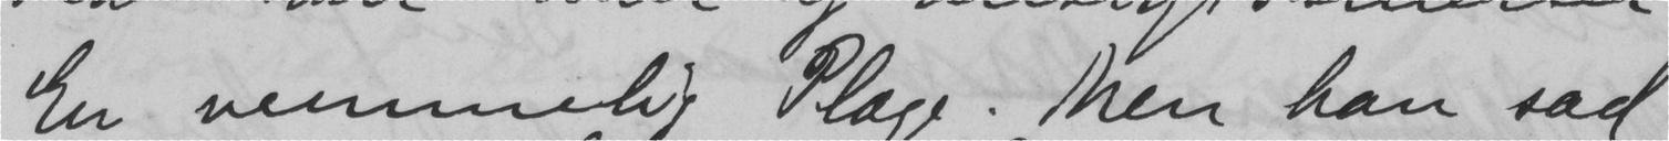En vemmelig plage. Men han sad
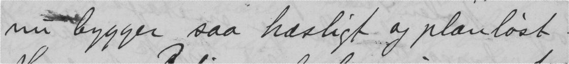nu bygger saa hæsligt og planløst.
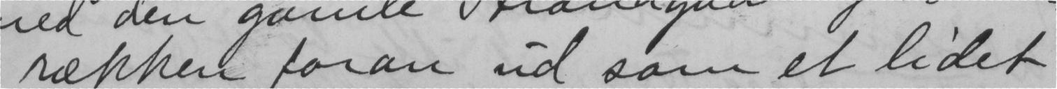rækken foran ud som et lidet
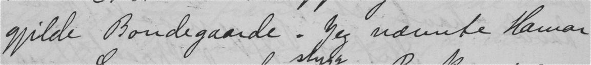gjilde Bondegaarde. Jeg nævnte Hamar
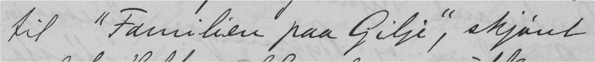til "Familien paa Gilje", skjønt
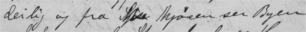deilig og fra  Mjøsen ser Byen
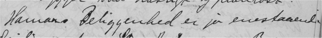Hamars Teliggenhed er jo enestaaende
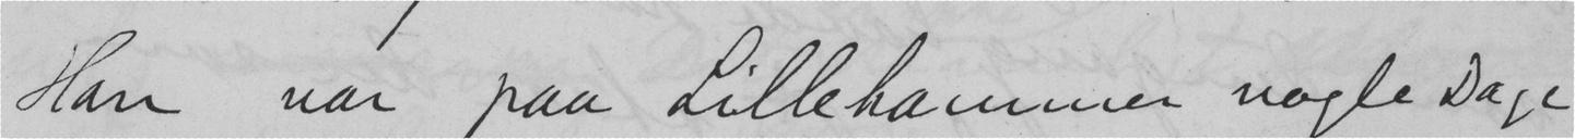Han var paa Lillehammer nogle Dage
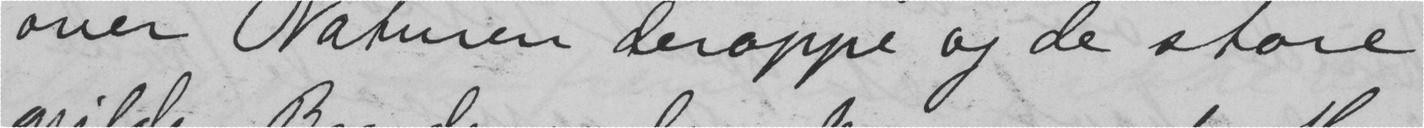over Naturen deroppe og de store
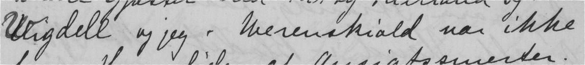Wigdell og jeg. Werenskiold var ikke
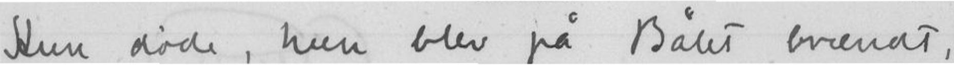Hun døde, hun blev på Bålet brendt,
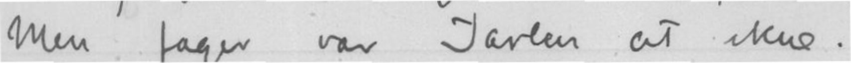Men fager var Jarlen at skue.
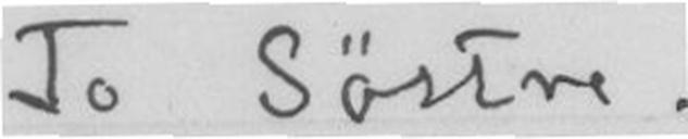To Søstre.
